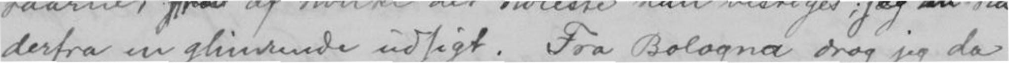derfra en glimrende ugsigt. Fra Bologna drog jeg da
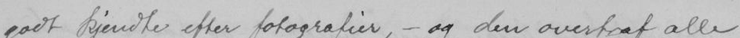godt kjendte efter fotografier, - og den overtraf alle
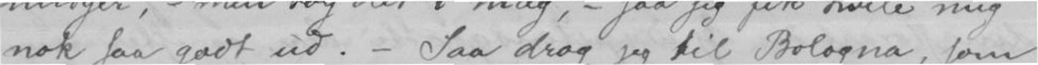nok saa godt ud. - Saa drogjeg til Bologna, som
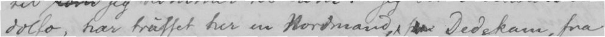dolfo, har truffet her en Nordmand, Dedekam, fra
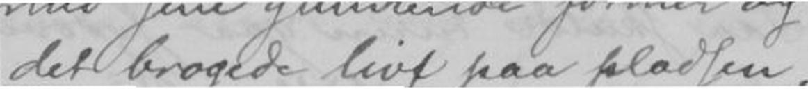det brogede liv paa pladsen,
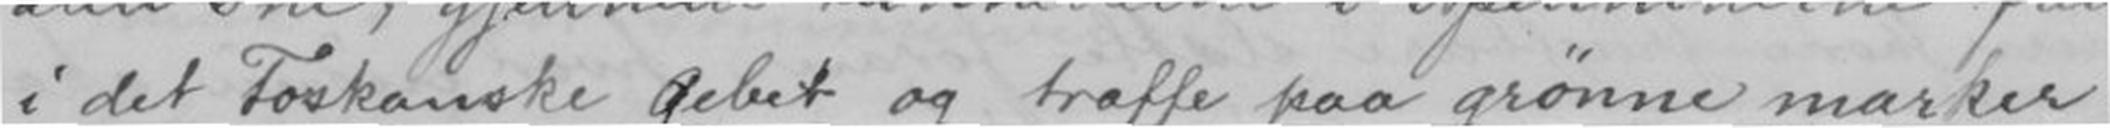i det toskanske gebet og træffe paa grønne marker
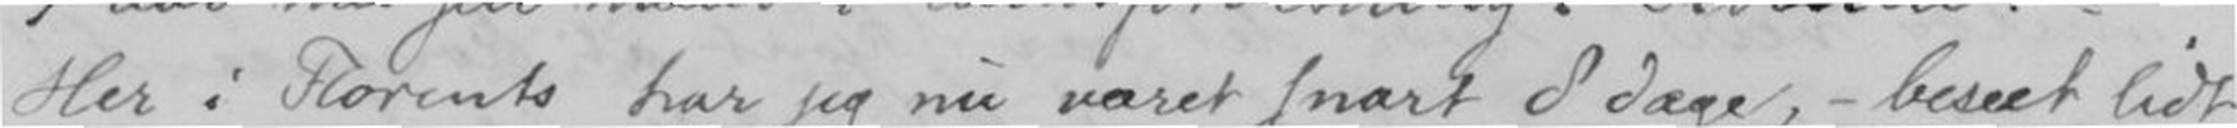Her i Florents har jeg nu været snart 8 dage, - beseet lidt
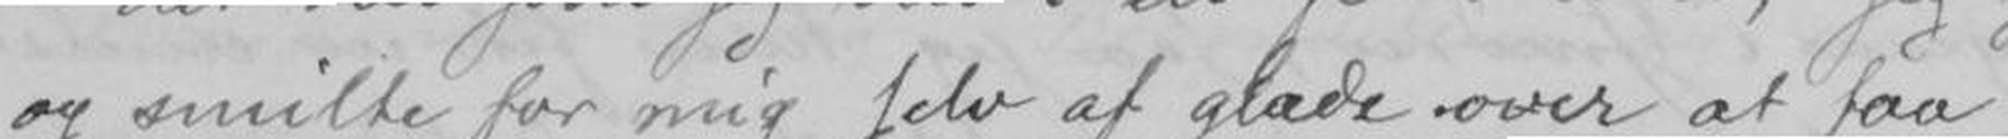og smilde for mig selv af glæde at faa
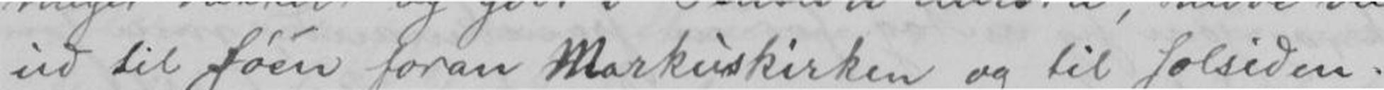ud til søen foran Markuskirken og til solsiden.
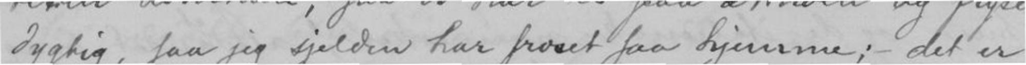dyktig, saa jeg sjelden har froset saa hjemme; - det er
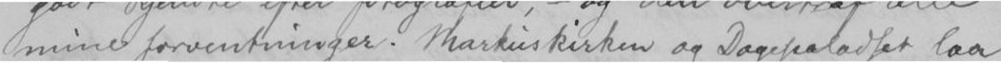mine forventninger. Markuskirken og Dogepaladset laa
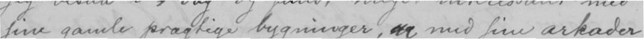fine gamle præktige bygninger, med fine arkader
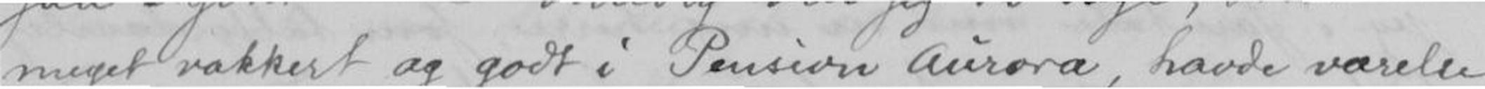meget vakkert og godt i Pension Aurora, havde værelse
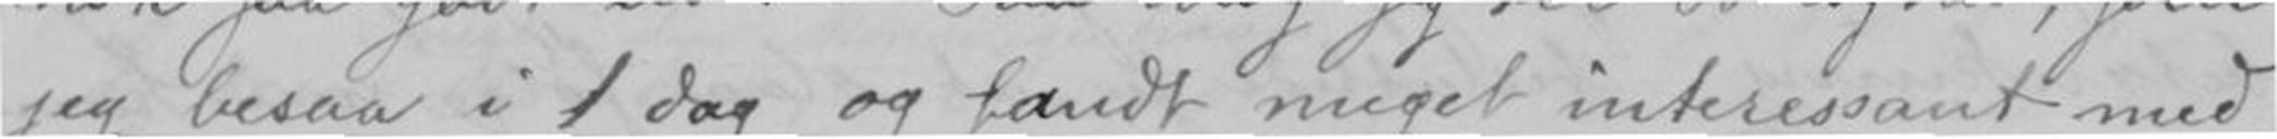jeg besaa i 1 dag og fandt meget interessant med
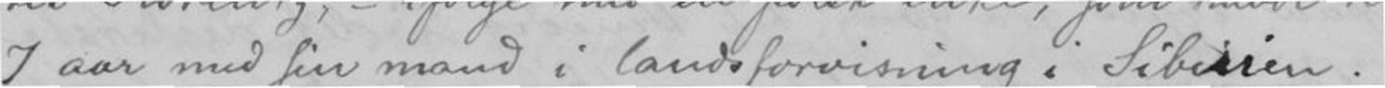7 aar med sin mand i landforvisning i Siberien. -
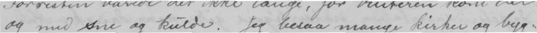og med sne og kulde. Jeg besaa mange kirker og byg-
